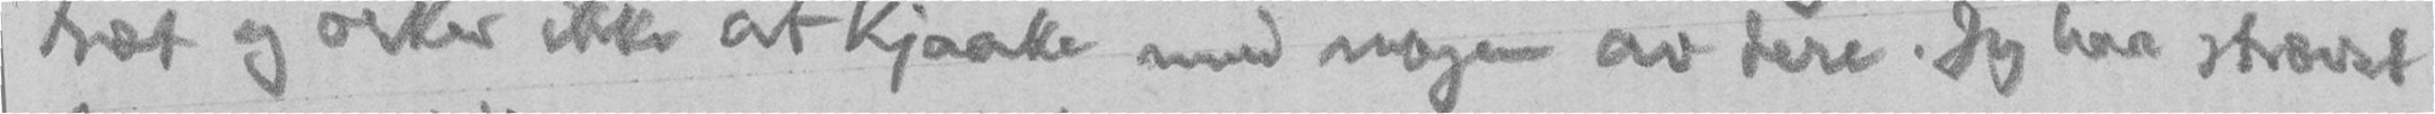træt og orker ikke at kjaake med nogen av dere. Jeg har strævet
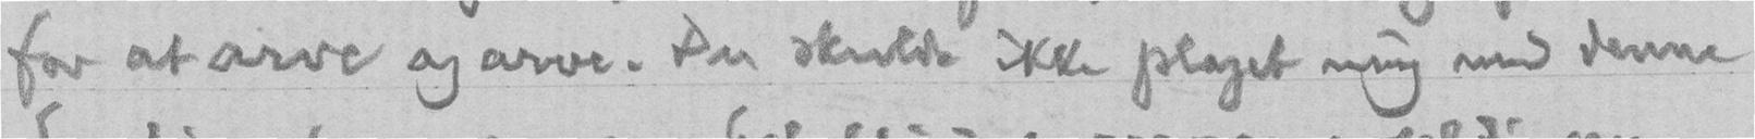for at arve og arve. Du skulde ikke plaget mig med denne
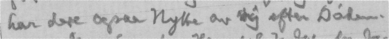har dere ogsaa Nytte av mig efter Døden.
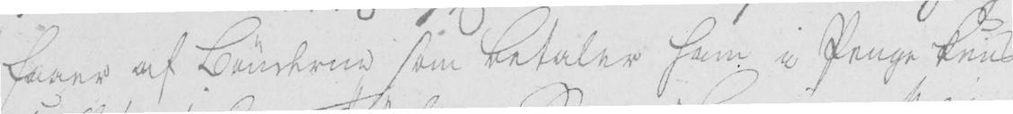faaer af Bønderne som betaler ham i Penge kund
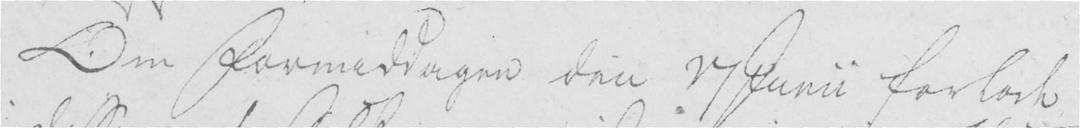Om Formiddagen den 17 Junii forlade
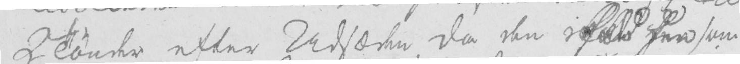2 Tønder efter Udsæden da den ikke her som
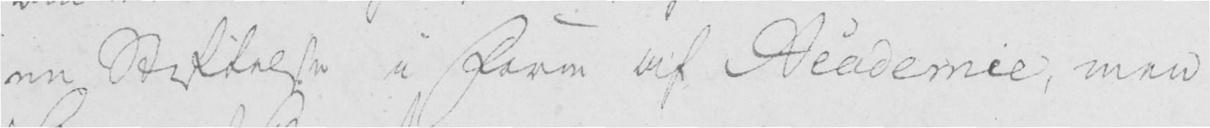en Stiftelse i Form af Academie, men
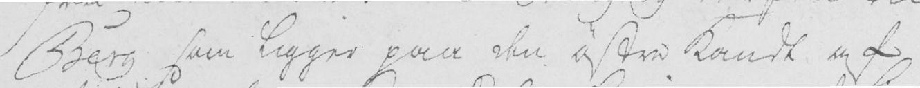Berg som ligger paa den østre Kandt af
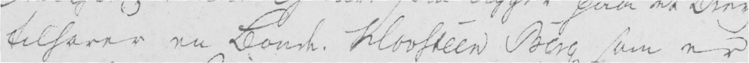tilhører en Bonde Klovsteen Berg som er
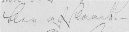blev afslaaet. -
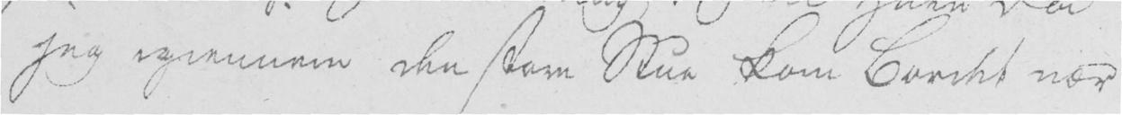jeg igiennem den store Stue kom Bordet nær
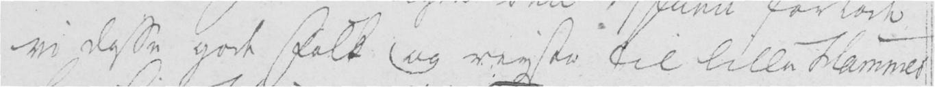vi disse godt Folk og reyste til lille Hammer
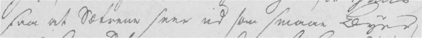saa at Sætrene seer ud som smaae Byer,
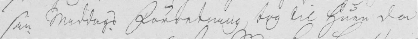sin Middags Forretning, tog til Huen da
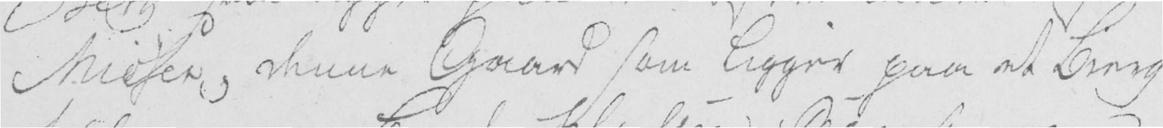Miøsen; denne Gaard som ligger paa et Bierg
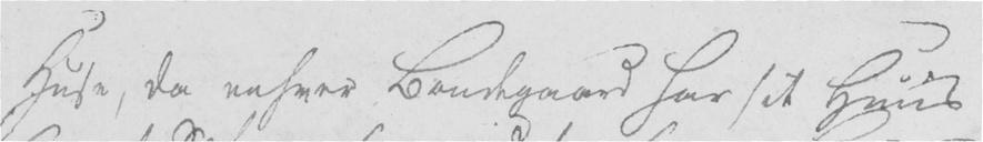Huse, da enhver Bondegaard har sit Huus
